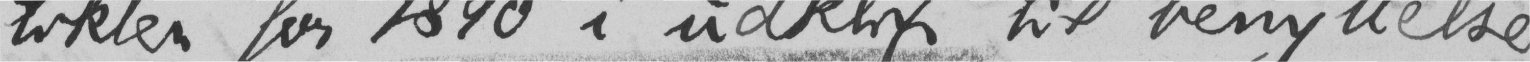tikler for 1890 i udklip til benyttelse.
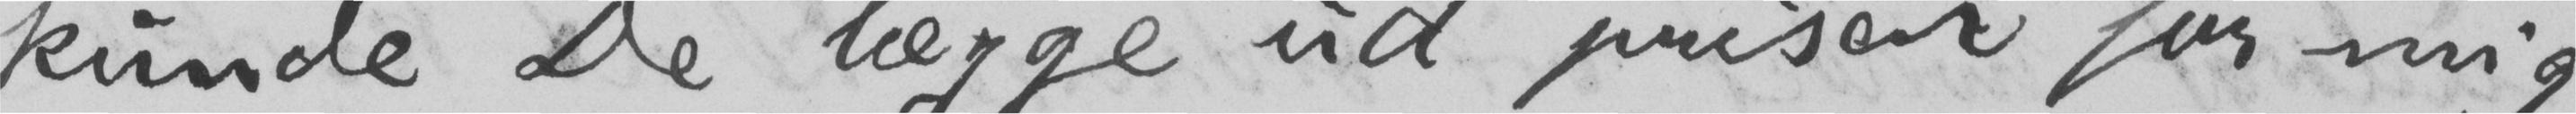kunde De lægge ud prisen for mig
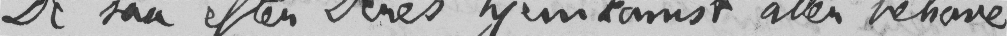De saa efter Deres hjemkomst atter behøve
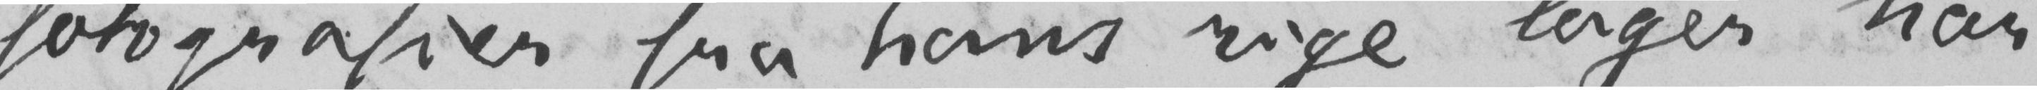fotografier fra hans rige lager har
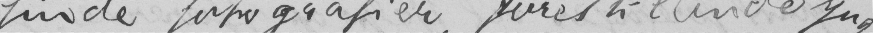finde fotografier, forestillende yng-
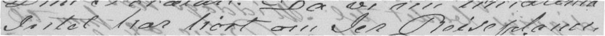Intet har hørt om Jer Reiseplaner,
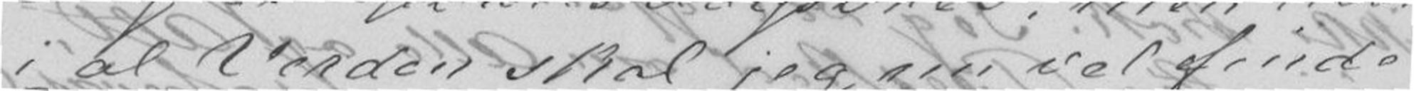i al Verden skal jeg nu vel finde
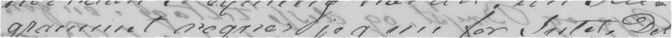grammet regner jeg nu for Intet Det
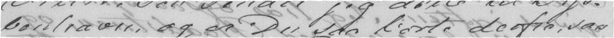benhavn, og er Du saa borte derfra saa
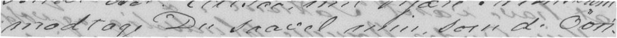modtag Du saavel min som de Øvri-
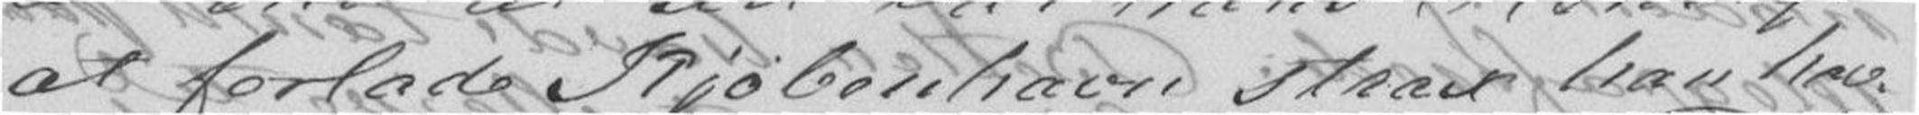at forlade Kjøbenhevn strax han hav-
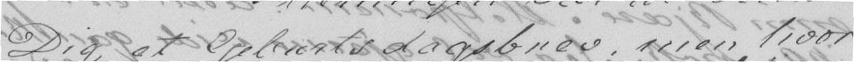Dig et Gebursdagsbrev; men hvor
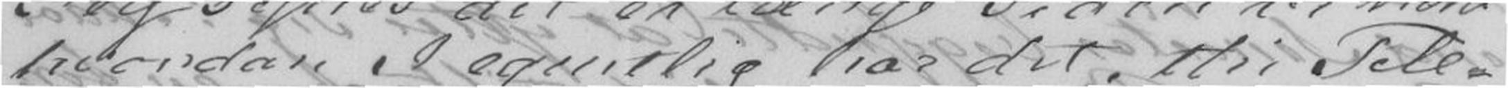hvordan I egentlig har det, thi Tele-
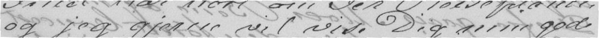og jeg gjerne vil vise Dig min gode
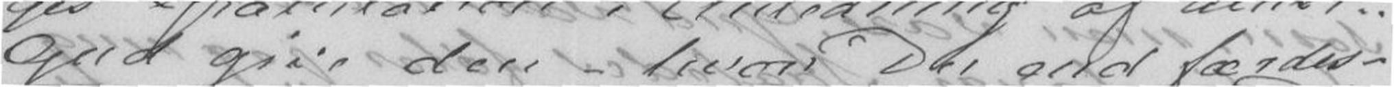Gud give den - hvor Du end færdes -
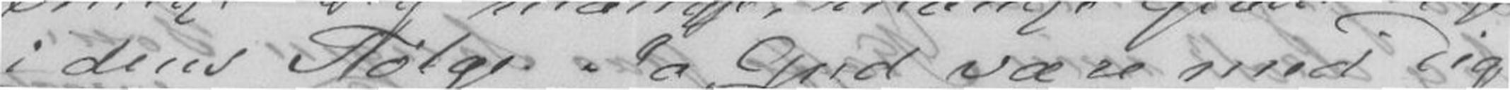i dens Følge. Ja Gud være med Dig
